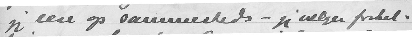jeg lese op sammesteds - jeg velger fortel-
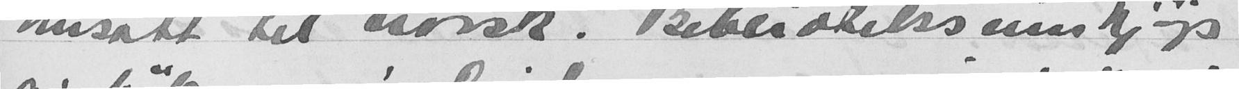omsatt til norsk. Bibliotekets innkjøp
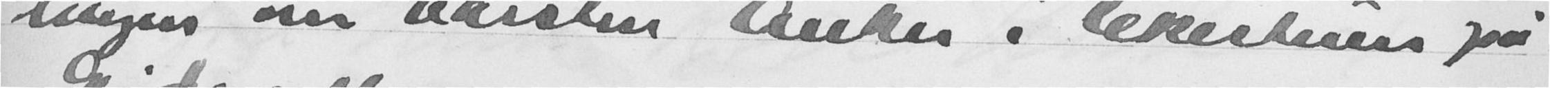lingen om Karsten Anker i lekestuen på
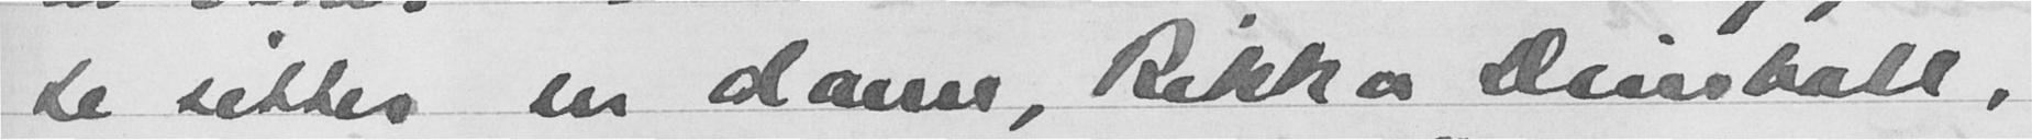te sitter en dame, Rikka Deinboll,
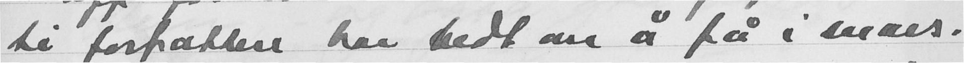ti forfattere har bedt om å få i mars.
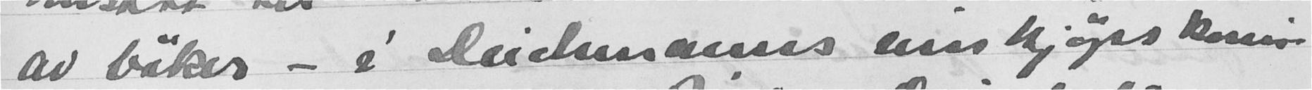av bøker - i Deichmanns innkjøps komi-
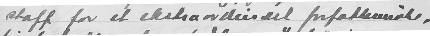stoff for et ekstraodinært forfattermøte,
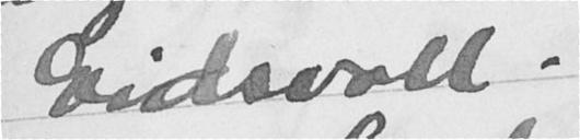Eidsvoll.
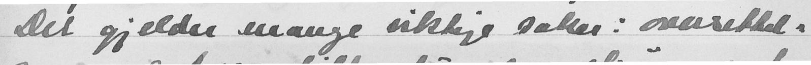Det gjelder mange viktige saker: oversettel-
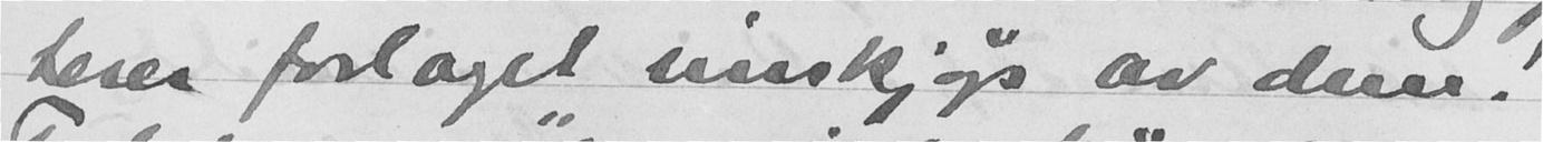terer forlaget innkjøp av dem.
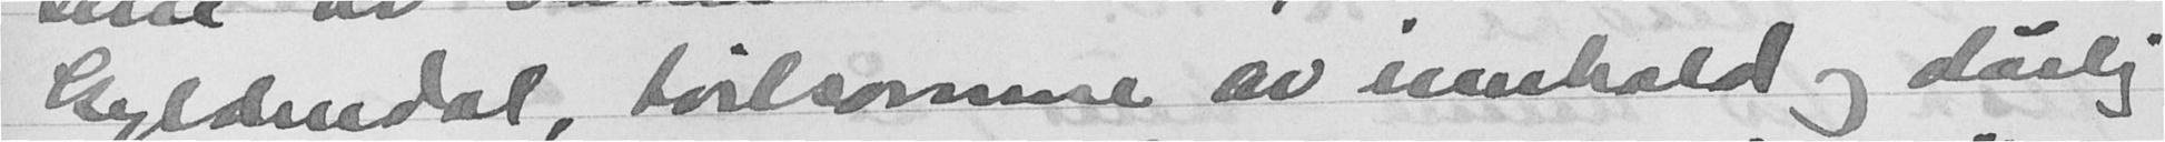Gyldendal, tvilsomme av innhold og dårlig
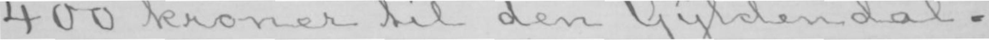400 kroner til den Gyldendal-
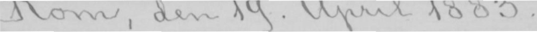Rom, den 19. April 1883.
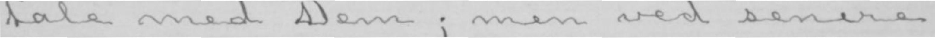tale med Dem; men ved senere
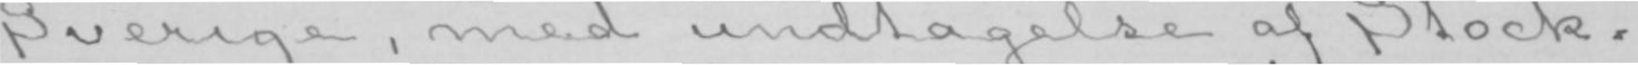Sverige, med undtagelse af Stock-
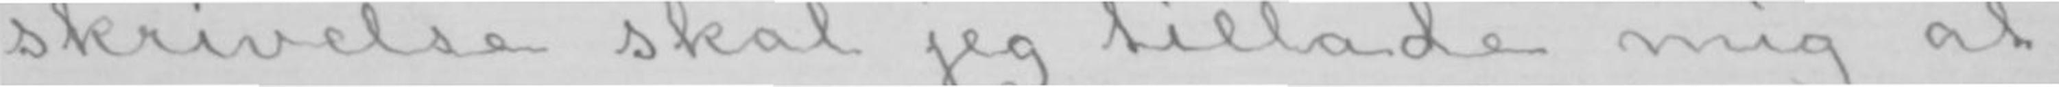skrivelse skal jeg tillade mig at
